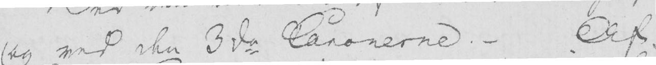og ved den 3de Canonerne. - Af
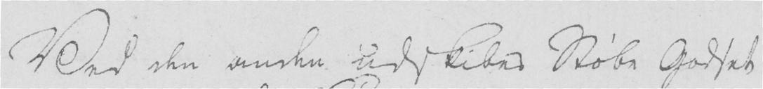Ved den anden udskibes Støbe Godset
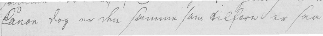Canon dog er den samme som tilforn er saa
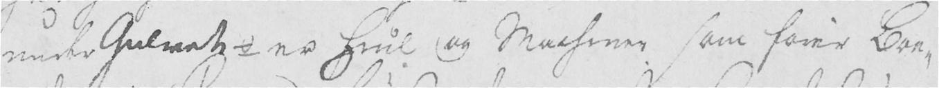under Gulvet er Hiul og Machiner som fører Boe-
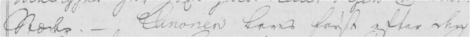Stæder. - Canonen bores først efter den
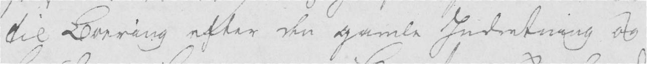til Boering efter den gamle Indretning og
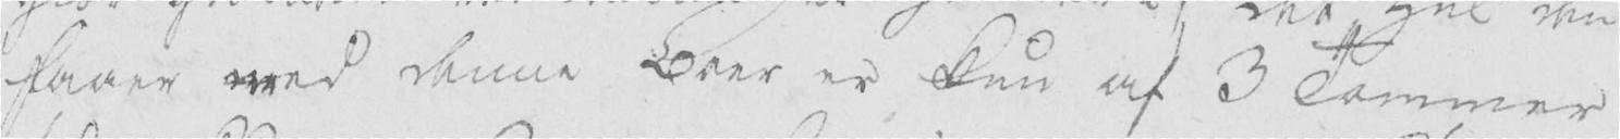faaer ved denne Boer er kun af 3 Tommer
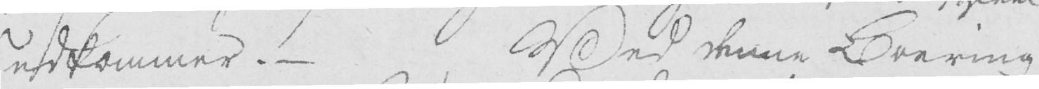udkommer. - Ved denne Boering
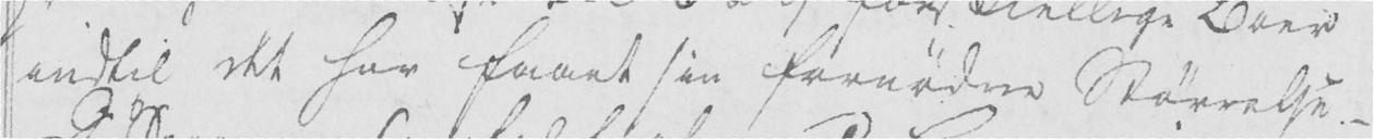indtil det har faaet sin fornødne Størrelse. -
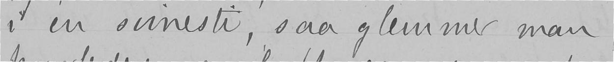i en svinesti, saa glemmer man,
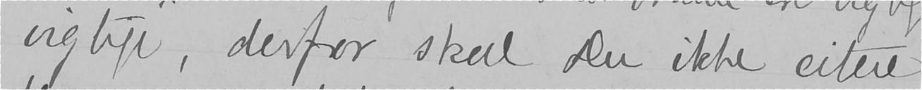vigtige, derfor skal Du ikke citere
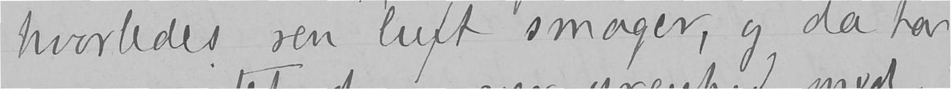hvorledes ren luft smager, og da har
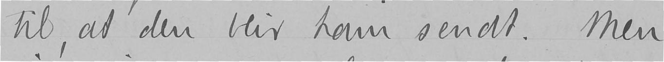til, at den blir ham sendt. Men
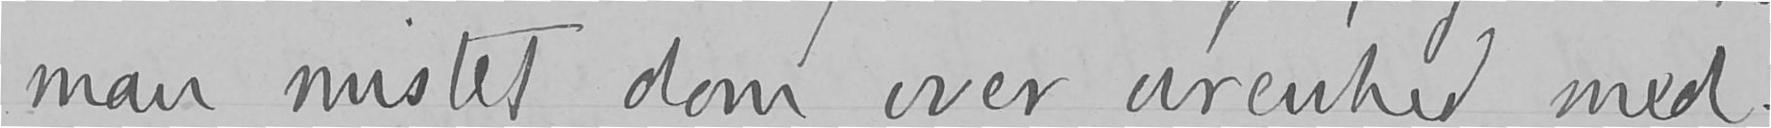man mistet dom over urenhed med.
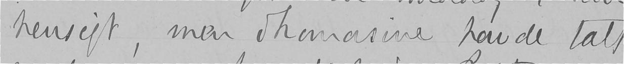hensigt, men Thomasine havde talt
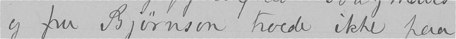og fru Bjørnson troede ikke paa
